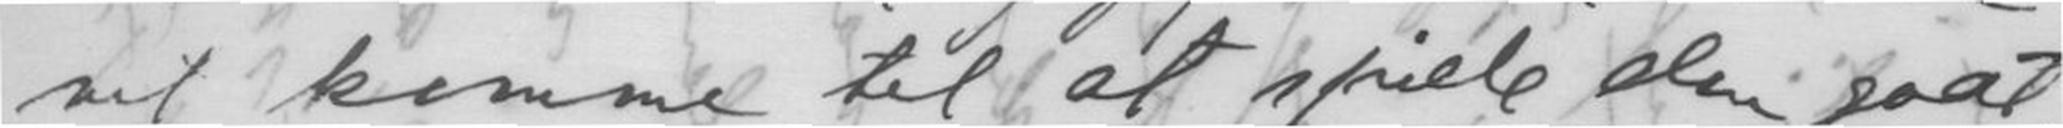vil komme til at spille den godt.
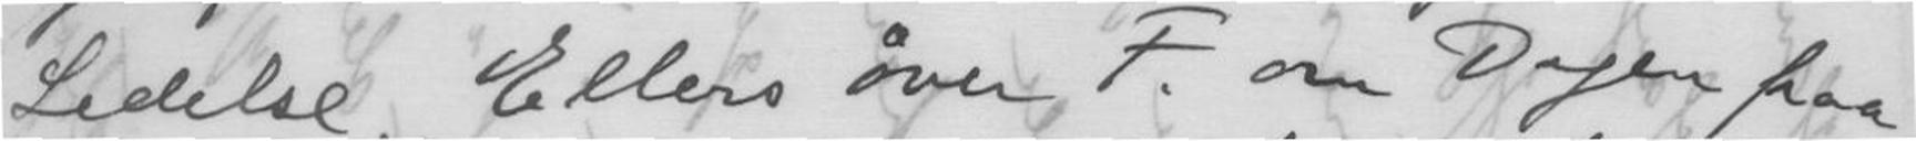Ledelse. Ellers øver F. om Dagen paa
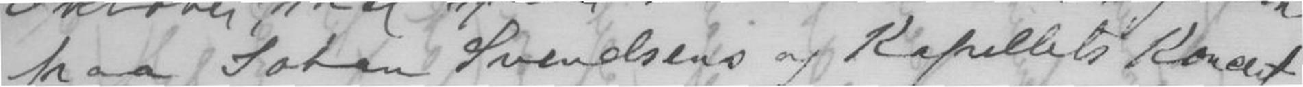paa Johan Svendsens og Kapellets Koncert.
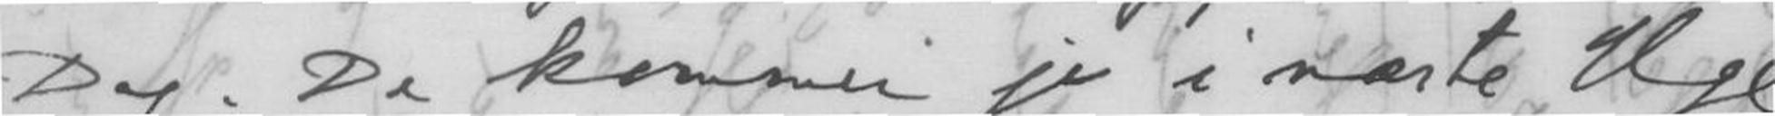Dag. De kommer jo i næste Uge
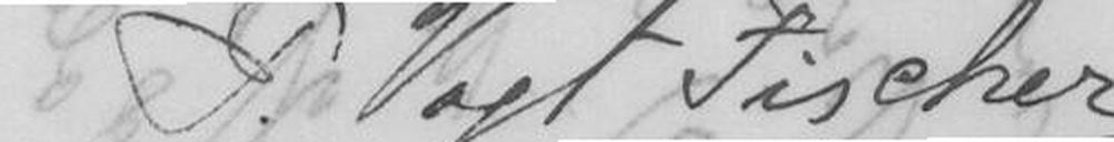P. Vogt Fischer
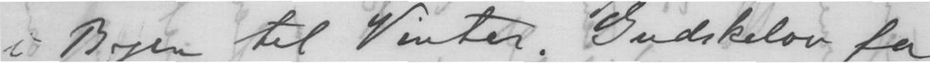i Byen til Vinter. Gudskelov for
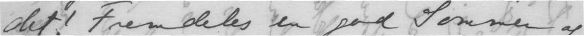det! Fremdeles en god Sommer og
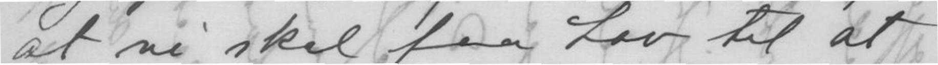at vi skal faa Lov til at
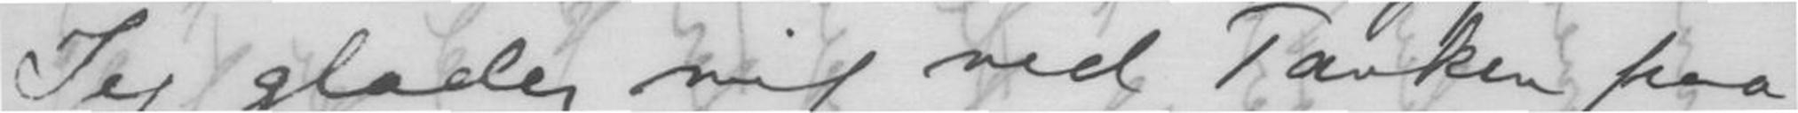Jeg glæder mig ved Tanken paa
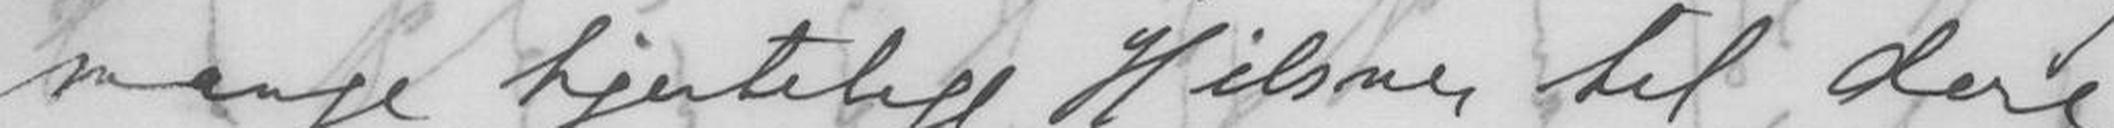mange hjertelige Hilsner til dere
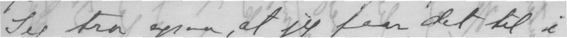Jeg tror ogsaa, at jeg faar det til i
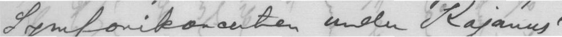Symfonikoncerten under Kajanus'
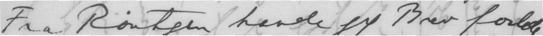Fra Röntgen havde jeg Brev forleden
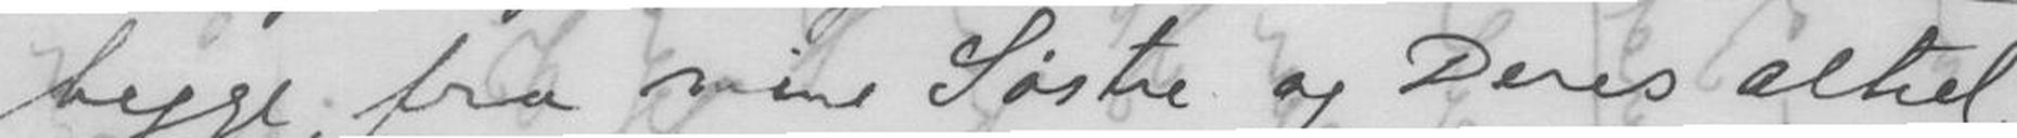begge, fra mine Søstre og Deres Altid
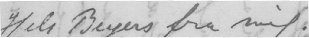Hils Beyers fra mig.
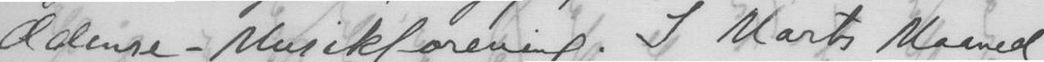Odense-Musikforening. I Marts Maaned
